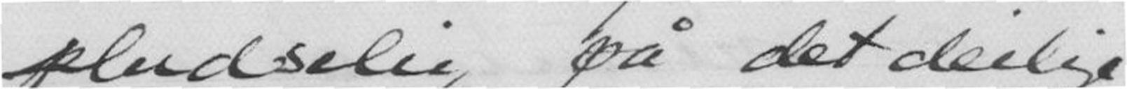pludselig på det deilige
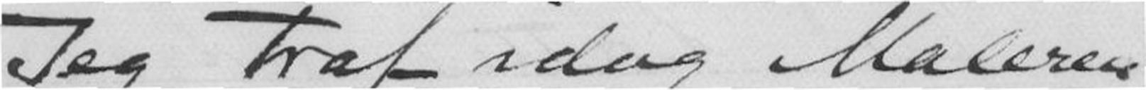Jeg traf idag Maleren
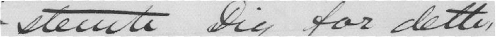stemte Dig for dette,
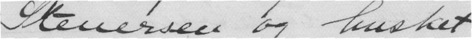Stenersen og husket
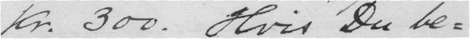Kr. 300. Hvis Du be-
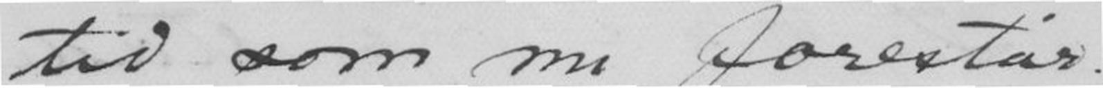tid som nu forestår.
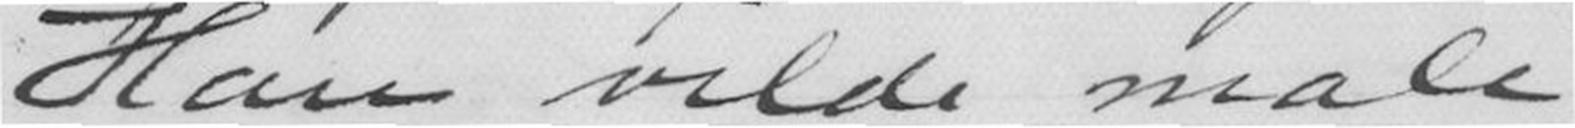Han vilde male
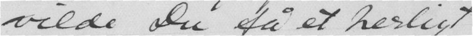vilde Du få et herligt
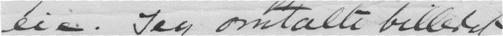eie. Jeg omtalte billedet
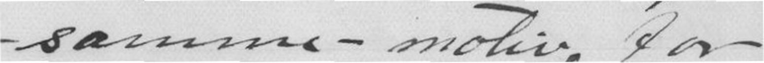- samme - motiv, for
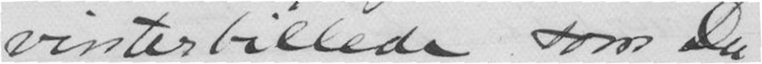vinterbillede som Du
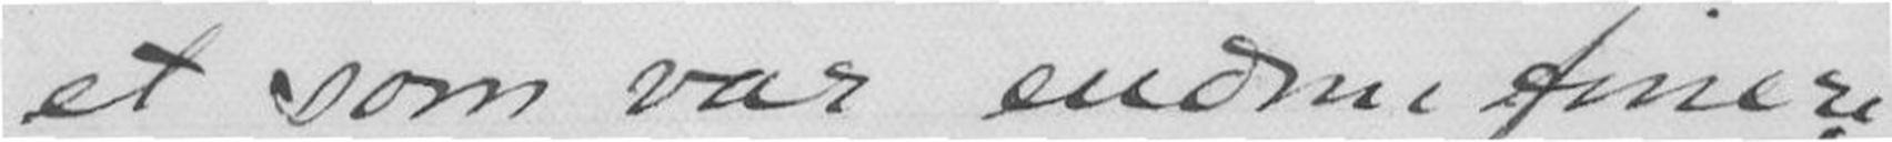et som var endnu finere,
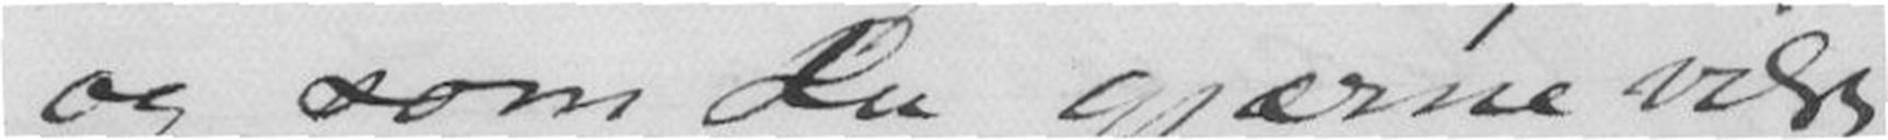og som Du gjærne vilde
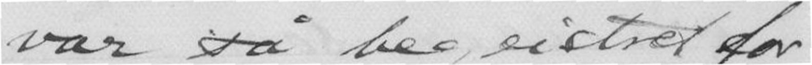var så begeistret for
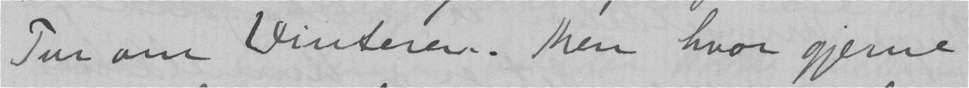Tur om Vinteren. Men hvor gjerne
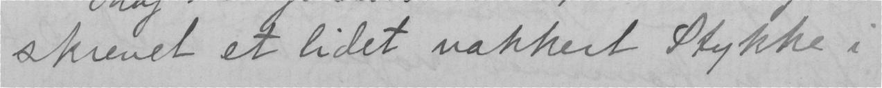skrevet et lidet vakkert stykke i
